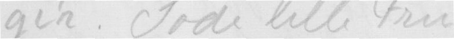gir. Søde lille Fru
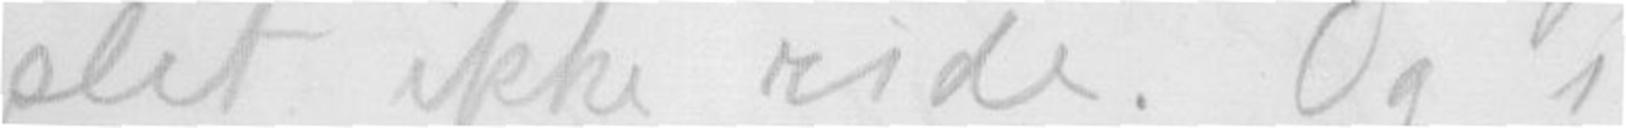slet ikke ride. Og i
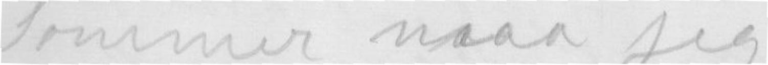Sommer naar jeg
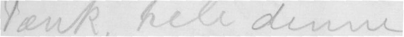Tænk, hele denne
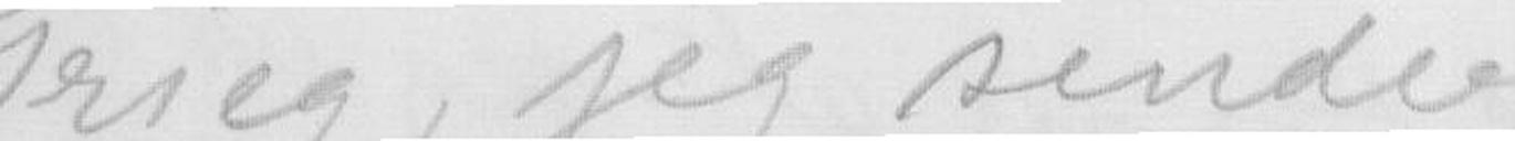Grieg, jeg sender
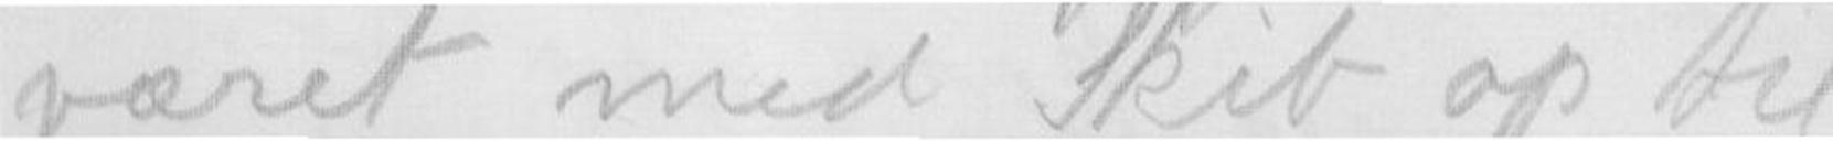været med Skib op til
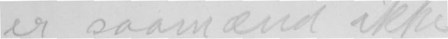7/ er saamænd ikke
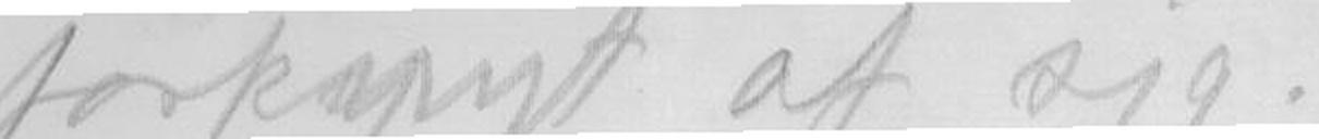forknyt af sig.
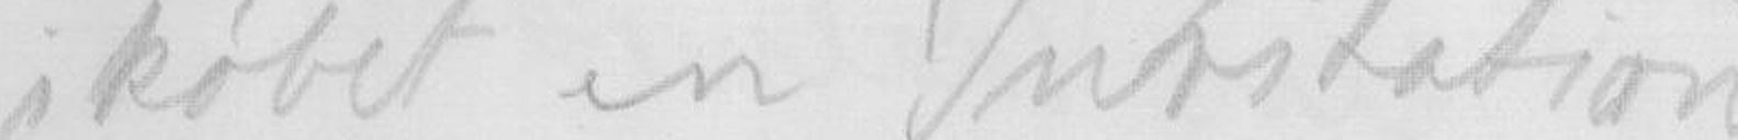ikøbet en Invitation.
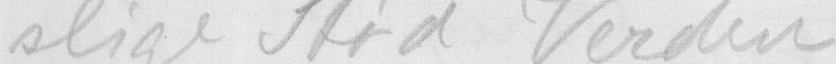slige Stød Verden
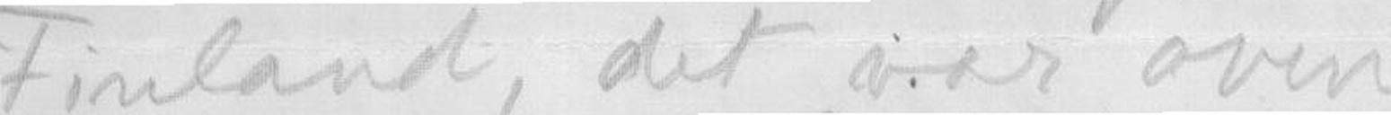Finland, det var oven-
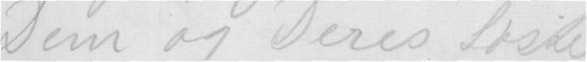Dem og Deres Søster
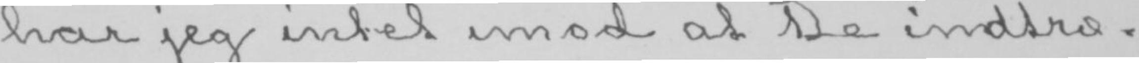har jeg intet imod at De imndtræ-
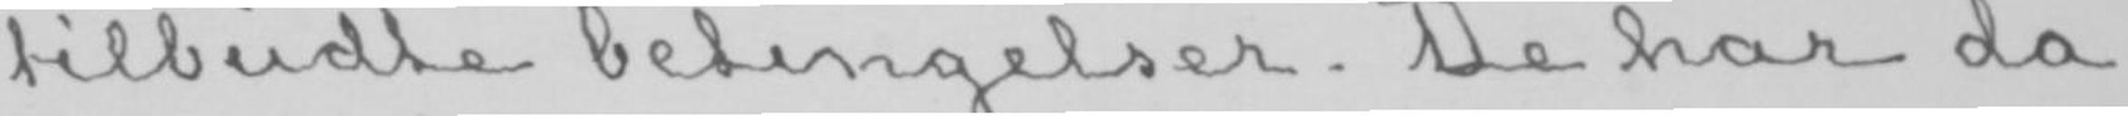tilbudte betingelser. De har da
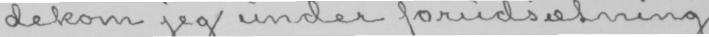dekom jeg under forudsætning
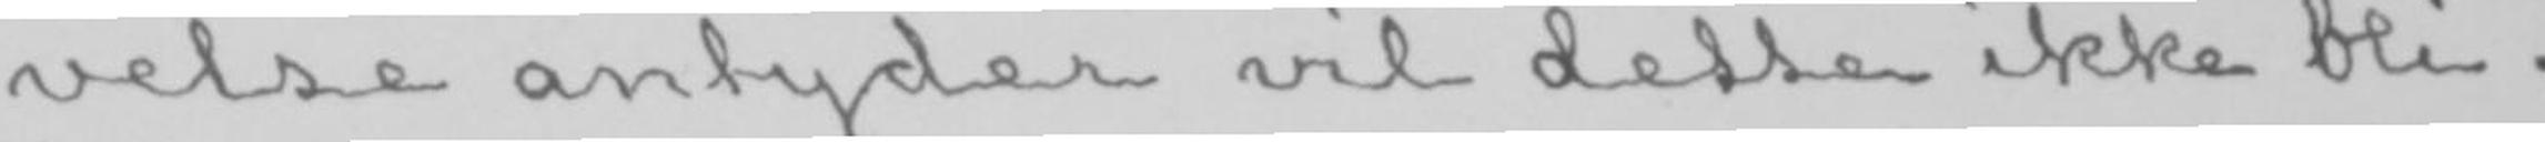velse antyder vil dette ikke bli-
